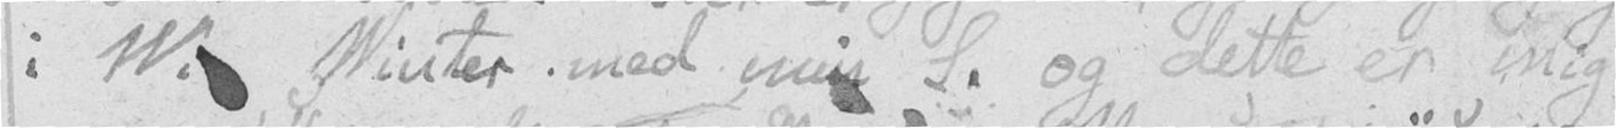i V. Vinter, med min S. og dette er mig
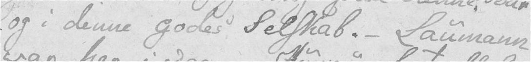og i denne godes Selskab. – Laumann
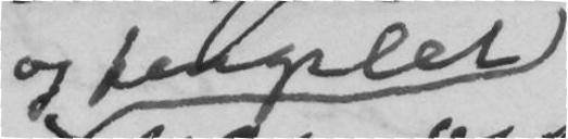og fengslet
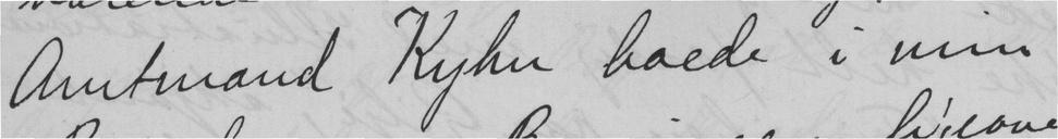Amtmand Kyhn boede i min
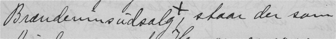Brændevinsudsalg, staar der som
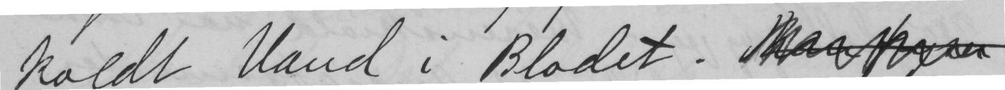koldt Vand i Blodet.
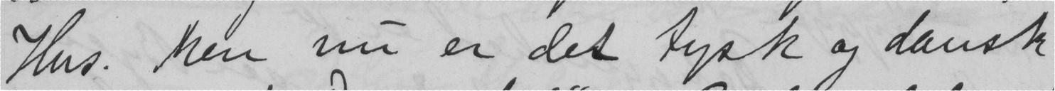Hus. Men nu er det tysk og dansk
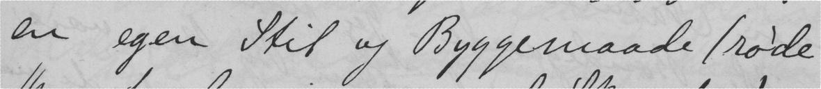en egen Stil og Byggemaade (røde
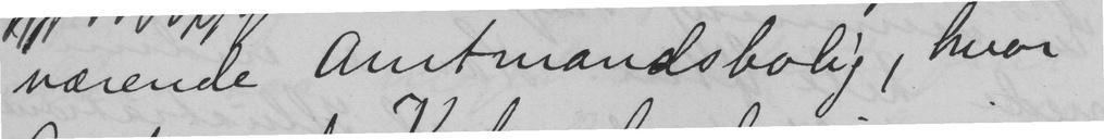værende Amtmandsbolig, hvor
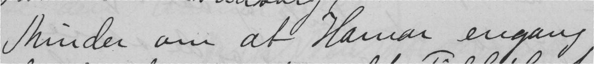minder om at Hamar engang
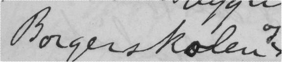Borgerskolen
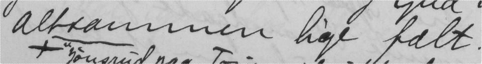altsammen lige fælt.
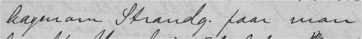bagenom Strandg. faar man
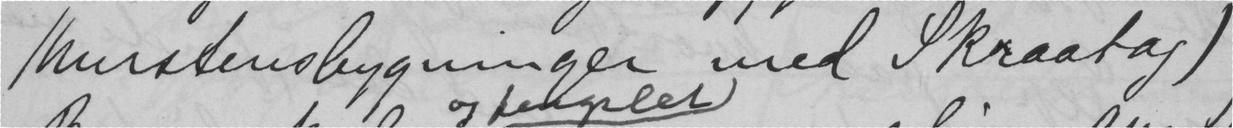Murstensbygninger med Skraatag)
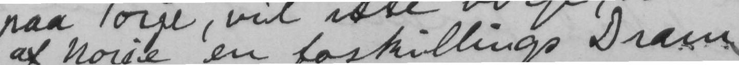af norje en toskillings Dram
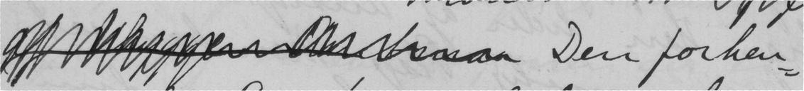Den forhen-
